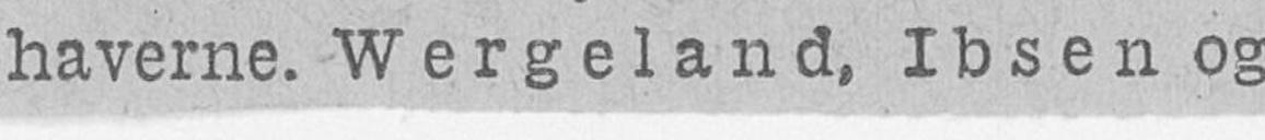haverne. Wergeland, Ibsen og
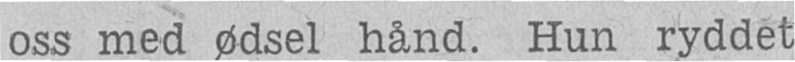oss med ødsel hånd. Hun ryddet
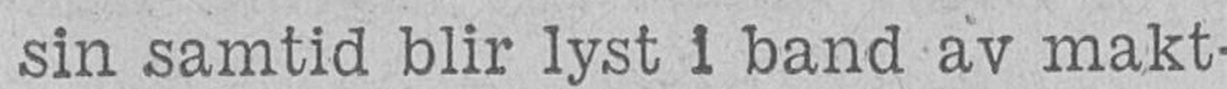sin samtid blir lyst i band av makt-
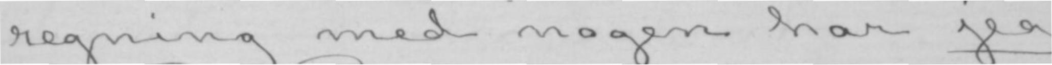regning med nogen har jeg
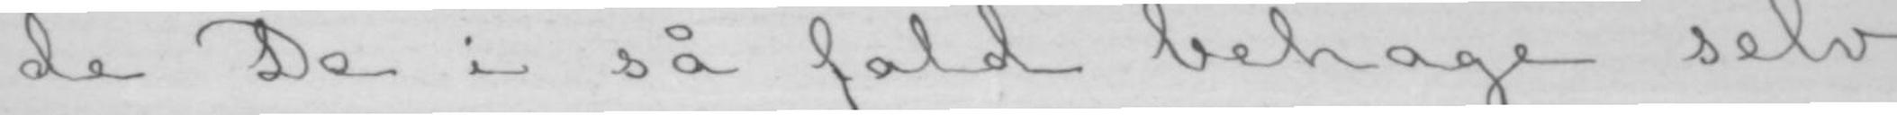de De i så fald behage selv
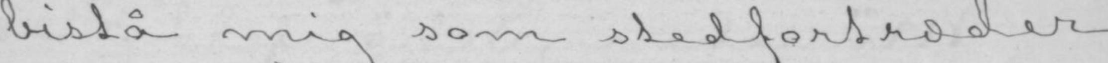bistå mig som stedfortræder
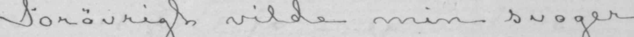Forøvrigt vilde min svoger,
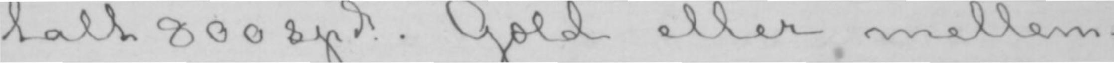talt 800 spd. Gæld eller mellem-
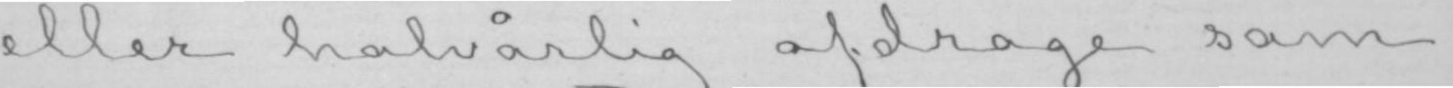eller halvårlig afdrage sam-
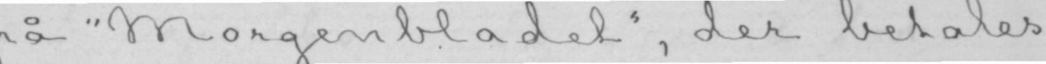på «Morgenbladet», der betales
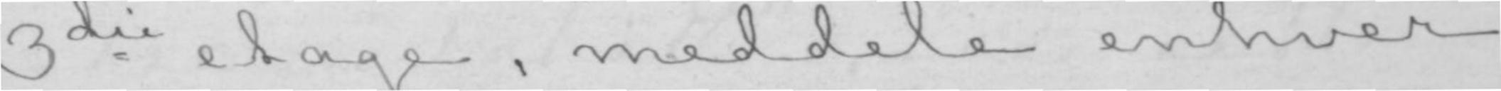3die etage, meddele enhver
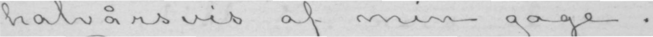halvårsvis af min gage.
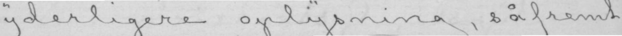yderligere oplysning, såfremt
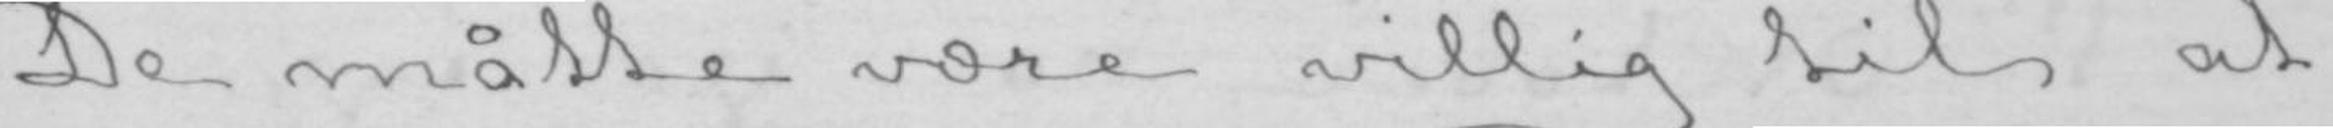De måtte være villig til at
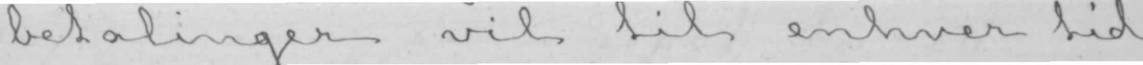betalinger vil til enhver tid
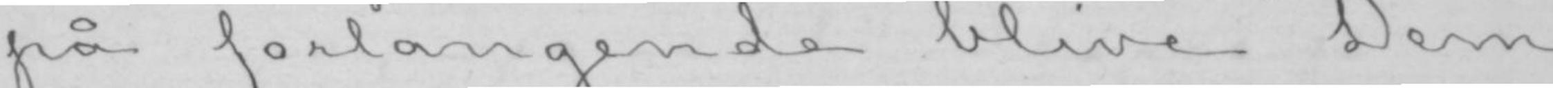på forlangende blive Dem
